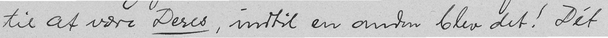til at være Deres, indtil en anden blev det! Dét
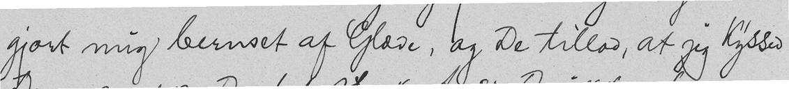gjort mig beruset af Glæde, og De tillod, at jeg kyssed
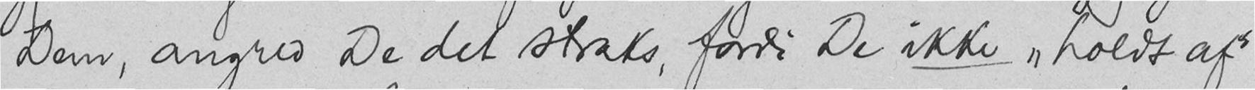Dem, angred De det straks, fordi De ikke "holdt af"
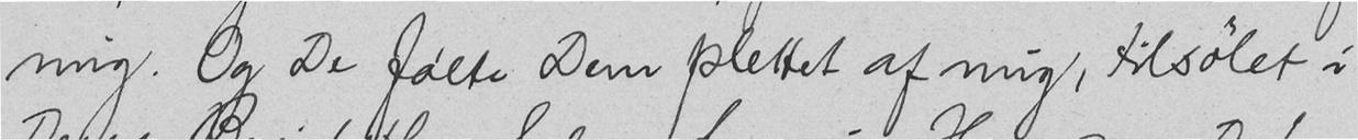mig. Og De følte Dem plettet af mig, tilsølet i
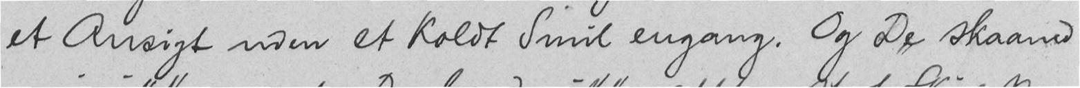et Ansigt uden et koldt Smil engang. Og De skaaned
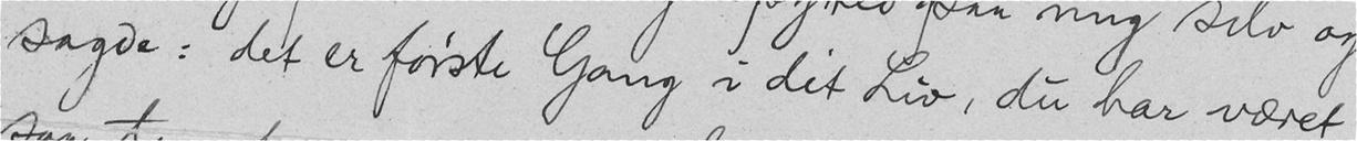sagde: det er første Gang i dit Liv, du har været
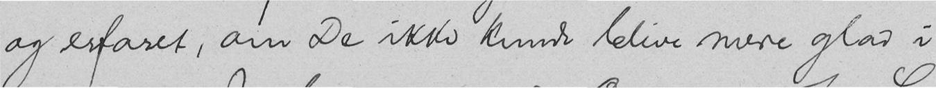og erfaret, om De ikke kunde blive mere glad i
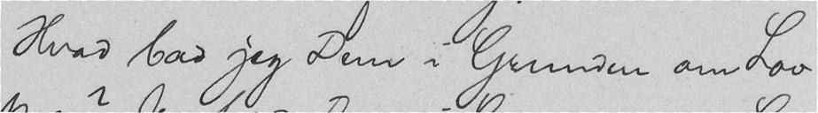Hvad bad jeg Dem i Grunden om Lov
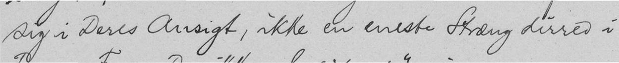sig i Deres Ansigt, ikke en eneste Stræng dirred i
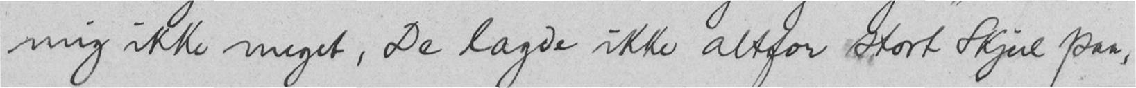mig ikke meget, De lagde ikke altfor stort Skjul paa,
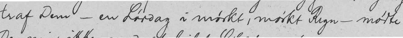traf Dem - en Lørdag i mørkt, mørkt Regn - mødte
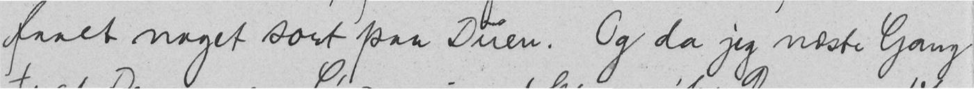faaet noget sort paa Duen. Og da jeg næste Gang
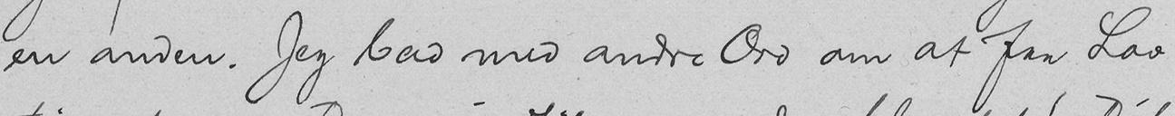en anden. Jeg bad med andre Ord om at faa Lov
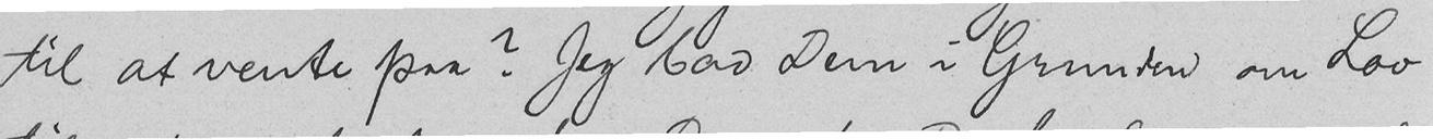til at vente paa? Jeg bad Dem i Grunden om Lov
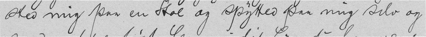sted mig paa en Stol og spytted paa mig selv og
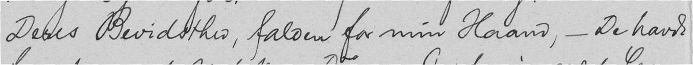Deres Bevidsthed, falden for min Haand, - De havde
